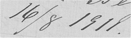16/8 1911.
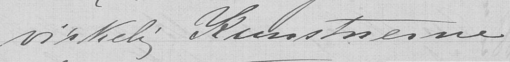virkelig Kunstnerne
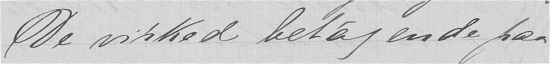De virkede betagende paa
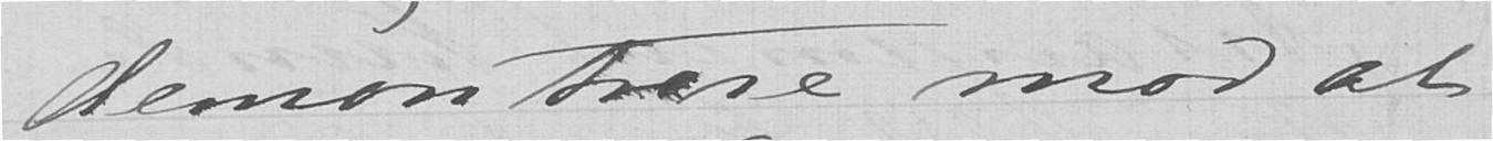demonstrere mod at
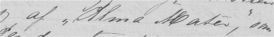og af  "Alma Mater", som
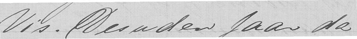Vis. Desuden faar da
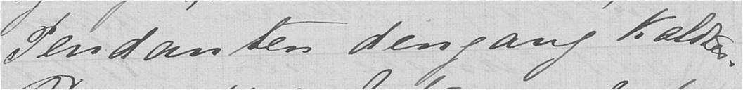Pendanten dengang kaldtes.
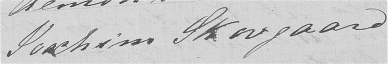Joachim Skovgaard
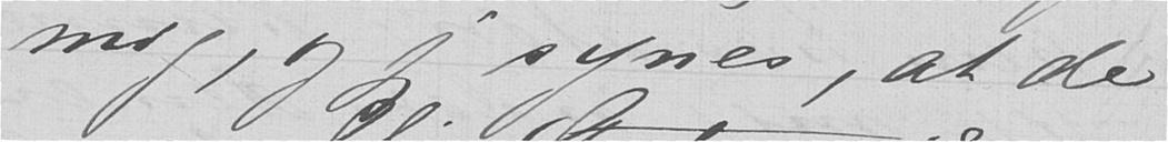mig, og jeg synes, at de
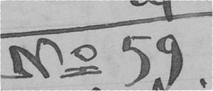No 59
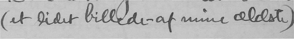(et lidet billede - af mine ældste)
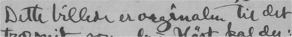Dette billede er orginalen til det
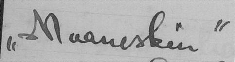"Maaneskin"
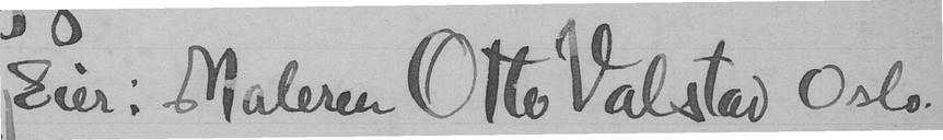Eier: Maleren Otte Valstad Oslo.
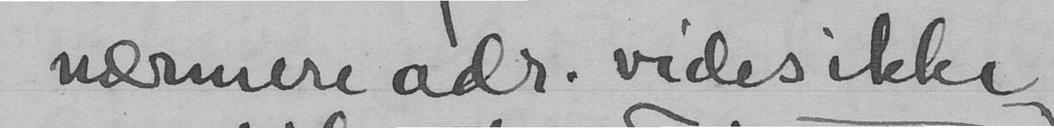nærmere adr. vides ikke
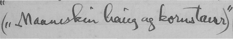("Maaneskin hang og kornstaur)"
